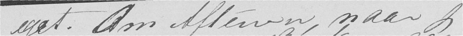eget. Om Aftenen, naar jeg
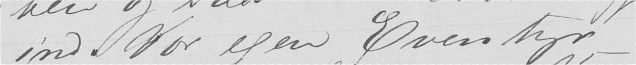ind. Vor egen Eventyr-
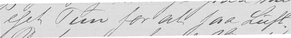eget Tun for at faa Luft.
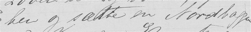ben og sætte en Nordbagge
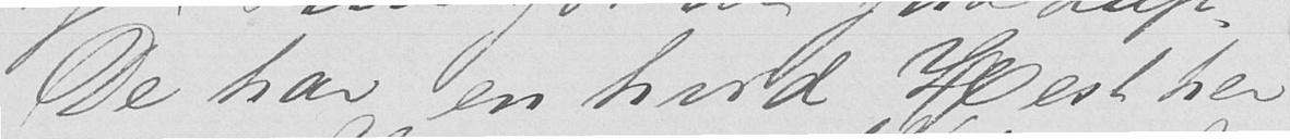De har en hvid Hest her
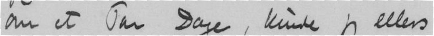om et Par Dage, kunde jeg ellers
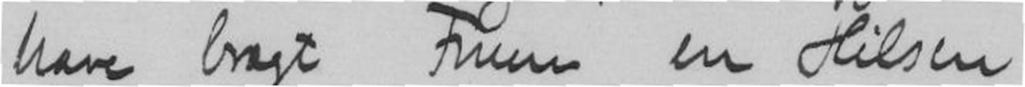have bragt Fruen en Hilsen
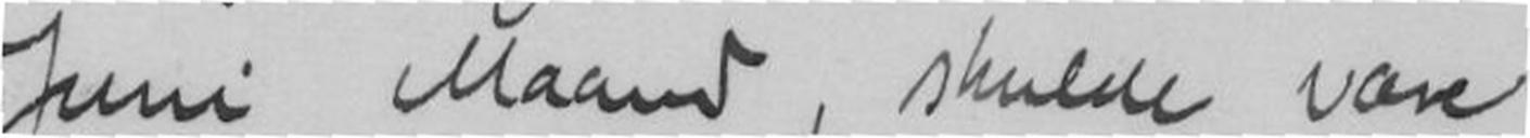Juni Maaned, skulde være
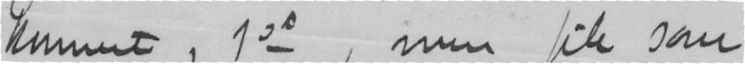kunnet, 1ste, men fik som
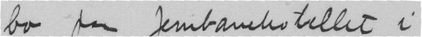bo paa Jernbanehotellet i
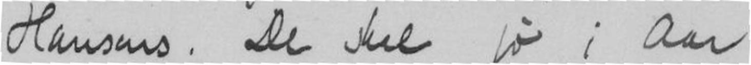Hansens. De skal jo i Aar
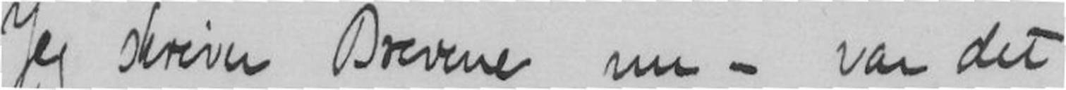Jeg skriver Brevene nu - var det
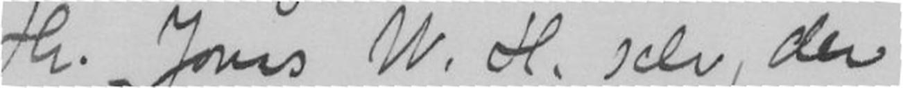Hr. Jonas W. H. selv, der
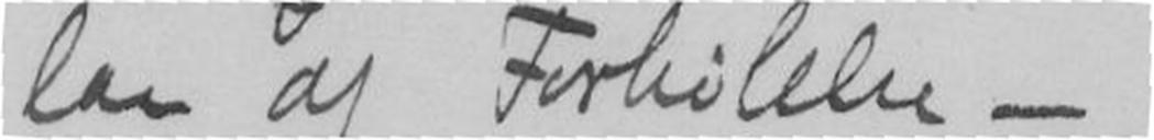laa af Forkølelse -
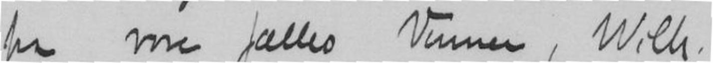fra vore fælles Venner, Wilh.
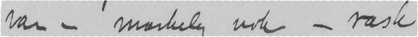var - mærkelig nok - rask.
